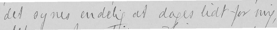det synes endelig at dages lidt for mig,
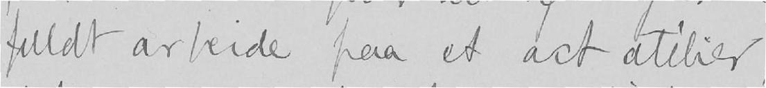fuldt arbeide paa et act atélier,
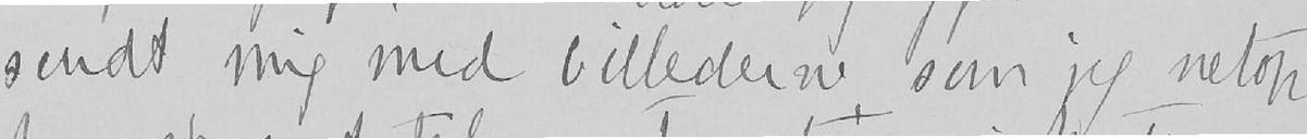sendt mig med billederne, som jeg netop
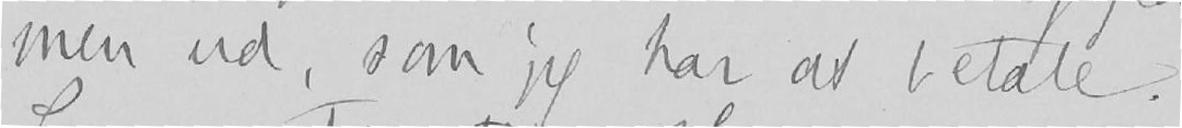men ud, som jeg har at betale.
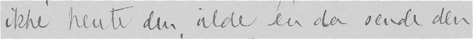ikke hente den, vilde Du da sende den
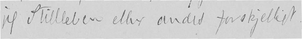jeg Stilleben eller andet forskjelligt.
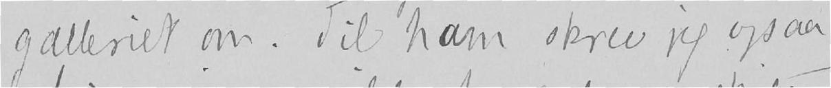galleriet om. Til ham skrev jeg ogsaa,
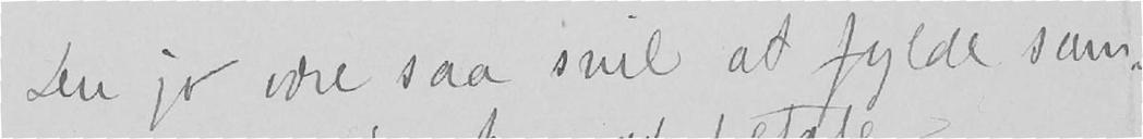Du jo være saa snil at fylde sum-
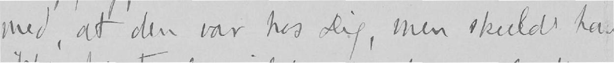med, at den var hos Dig, men skulde han
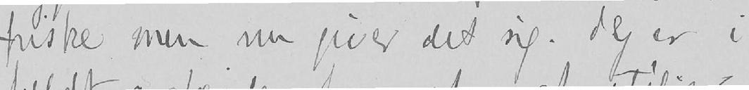friske men nu giver det sig. Jeg er i
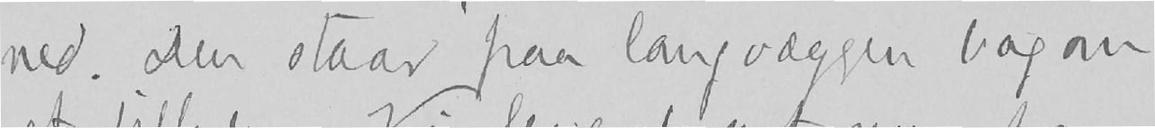ned. Den staar paa langvæggen bagom
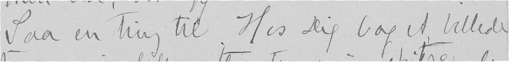Saa en ting til. Hos Dig bag et billede
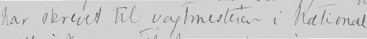har skrevet til vagtmesteren i National-
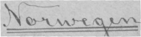Norwegen.
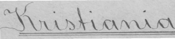Kristiania.
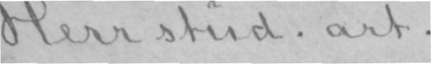Herr stud. art.
